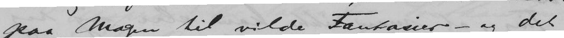paa Magen til vilde Fantasier - og det
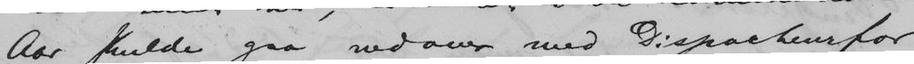Aar skulde gaa nedover med Dispacheurfor-
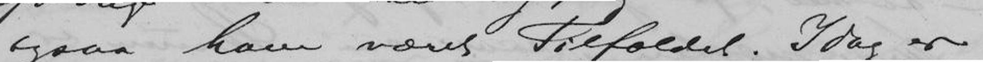ogsaa have været Tilfældet. Idag er
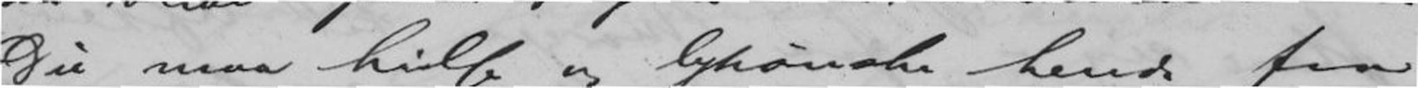Du maa hilse og lykønske hende fra
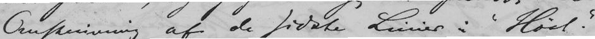Omskrivning af de sidste Linier i "Høst".
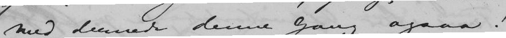med dernede denne Gang ogsaa!
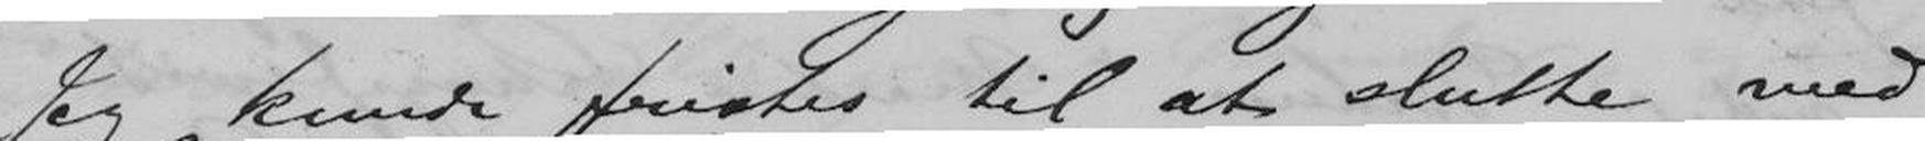Jeg kunde fristes til at slutte med
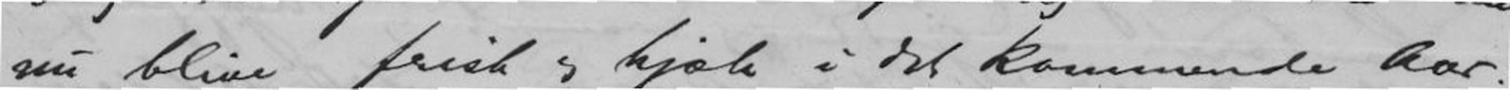nu blive frisk og kjæk i det kommende Aar!
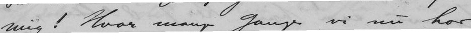mig! Hvor mange Gange vi nu har
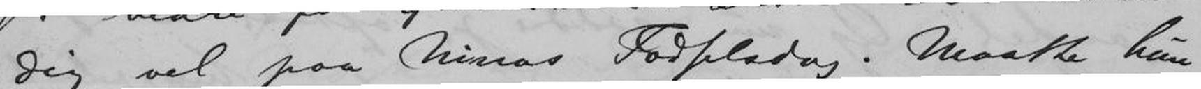dig vel paa Ninas Fødselsdag. Maatte hun
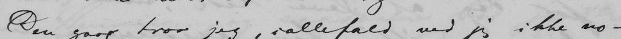Den gaar tror jeg, iallefald ved jeg ikke no-
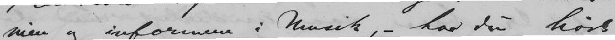nien og informere i Musik, - har du hørt
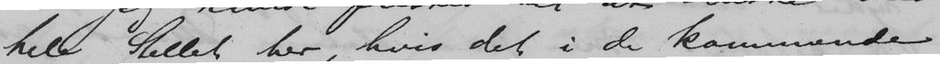hele Stellet her, hvis det i de kommende
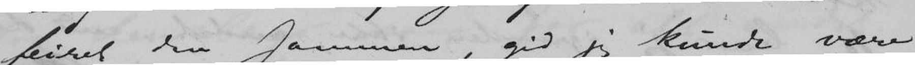feiret den sammen, gid jeg kunde være
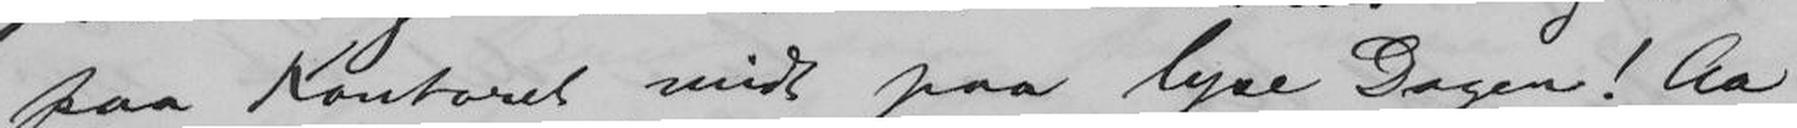paa Kontoret midt paa Lyse Dagen! Aa
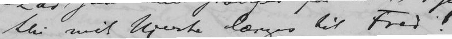thi mit Hjerte længes til Fred!"
